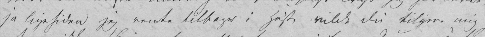ja ligesiden jeg vente tilbage i Høst vilde Du tilgive mig
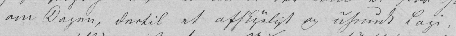om Dagen, dertil et afskyeligt og usundt Logi,
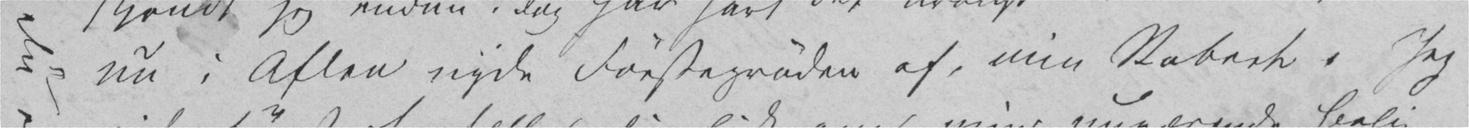nu i Aften nyde Førstegrøden af, min Robert. Jeg
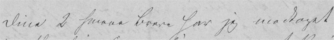Dine 2 smaae Breve har jeg modtaget
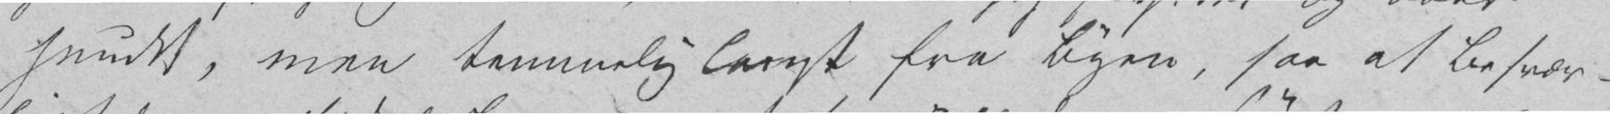sundt, men temmelig langt fra Byen, saa at
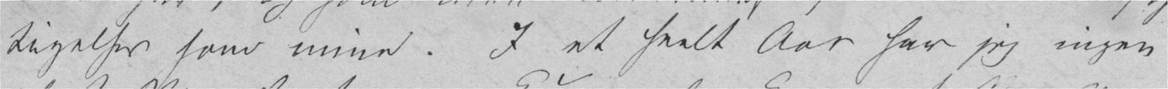tigelser som mine. I et heelt Aar har jeg ingen
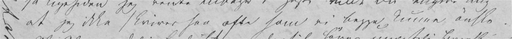at jeg ikke skriver saa ofte som vi begge kunne ønske.
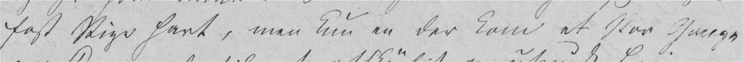fast Pige havt, men kun en der kom et Par Gange
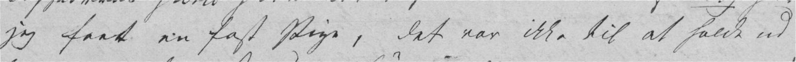jeg faaet en fast Pige, det var ikke til at holde ud
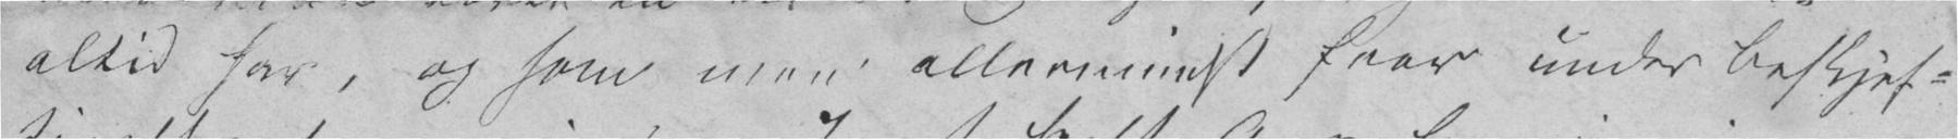altid har, og som man allermindst faar under Beskjef-
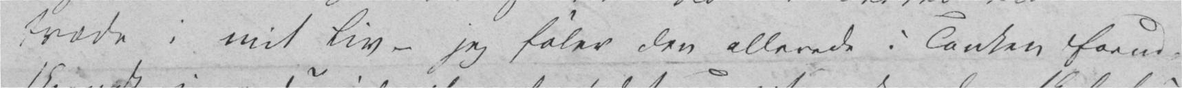træde i mit Liv – jeg føler den allerede i Tanken forud,
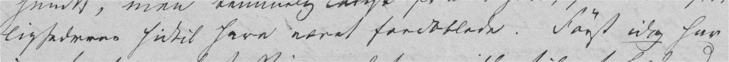Besværlighederne hidtil have været fordoblede. Først idag har
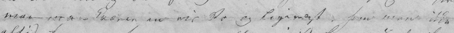maa man – kræver en viss Ro og Ligevægt, som man ikke
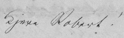Kjere Robert!
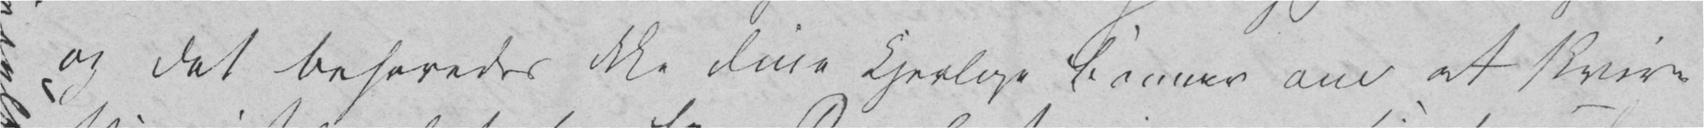og det behøvedes ikke Dine kjerlige Bønner om at skrive
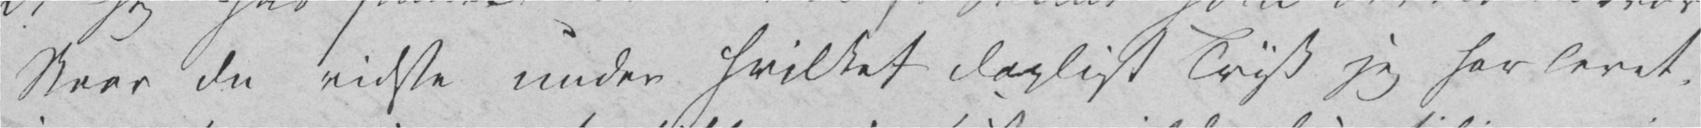Naar Du vidste under hvilket dagligt Tryk jeg har levet,
